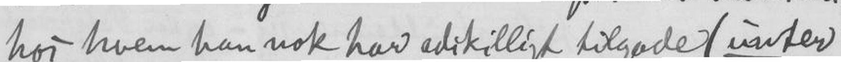hos hvem han nok har adskilligt tilgode (unter
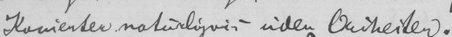Konserten naturligvis uden Orkester.
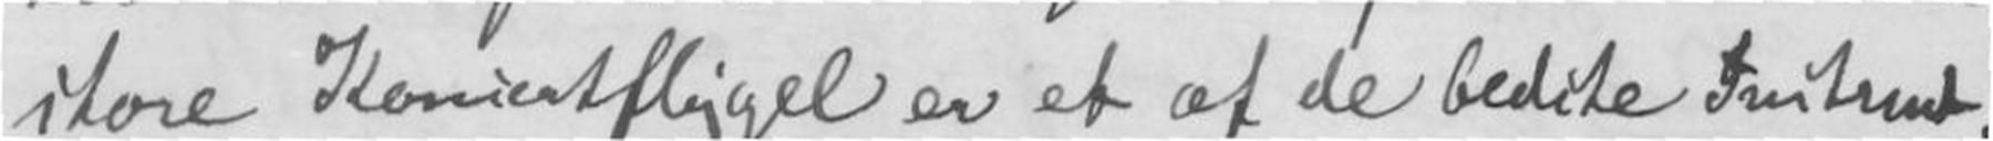store Koncertflygel er et af de bedste Instrumt.
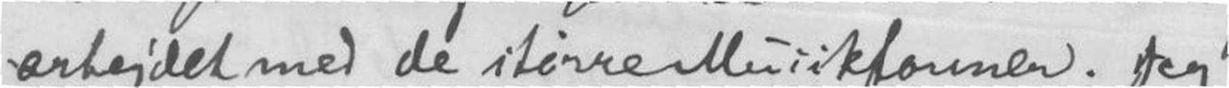arbejdet med de større Musikformer. Jeg
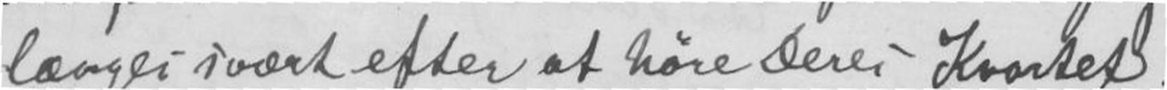længes svært efter at høre Deres Kvartet.
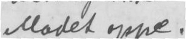Modet oppe.
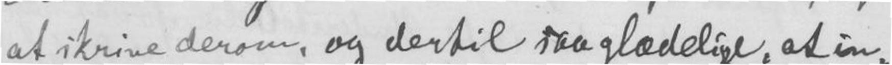at skrive derom, og dertil saa glædelige, at in-
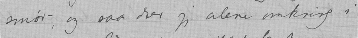smør —, og saa drev jeg alene omkring i
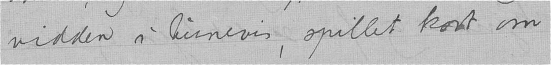vidden i timevis, spillet kort om
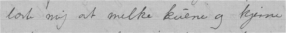lærte mig at melke kuene og kjerne
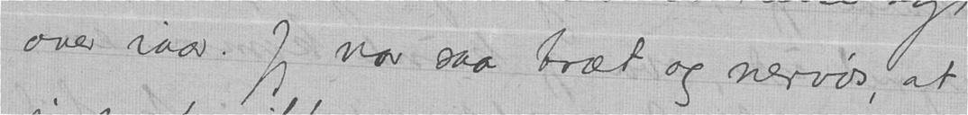over iaar. Jeg var saa træt og nervøs, at
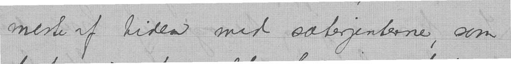meste af tiden med sæterjenterne, som
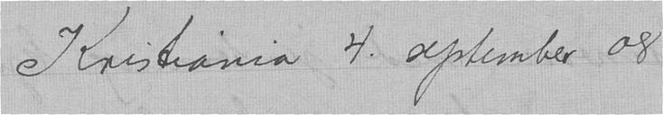Kristiania 4. september 08
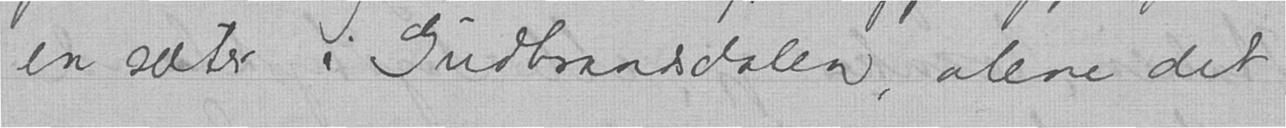en sæter i Gudbrandsdalen, alene det
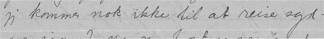jeg kommer nok ikke til at reise syd-
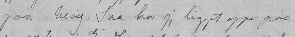paa besøg. Saa har jeg ligget oppe paa
